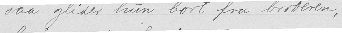saa glider hun bort fra broderen,
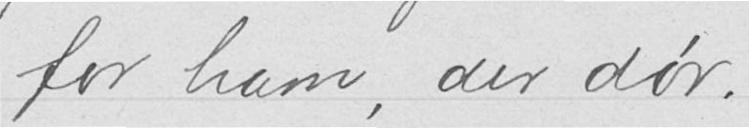for ham, der dør.
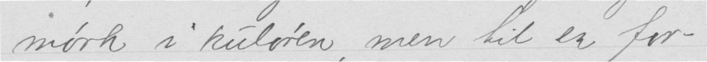mørk i kuløren, men til en for-
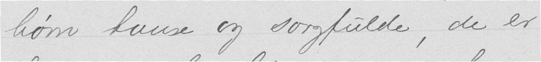børn tause og sorgfulde, de er
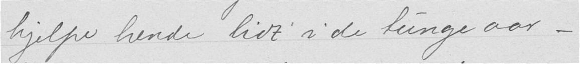hjelpe hende lidt i de tunge aar -
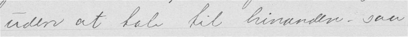uden at tale til hinanden - saa
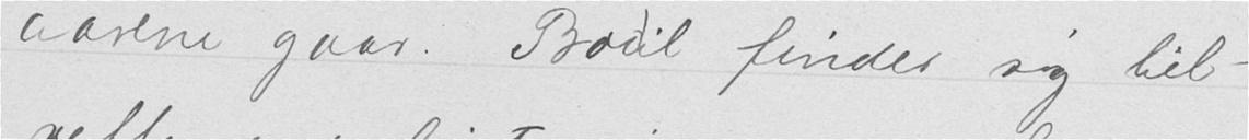aarene gaar. Bodil finder sig til-
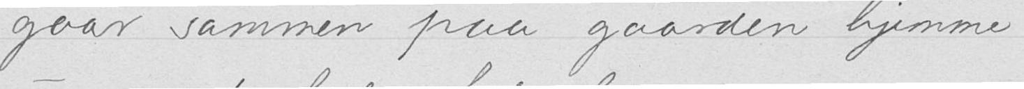gaar sammen paa gaarden hjemme
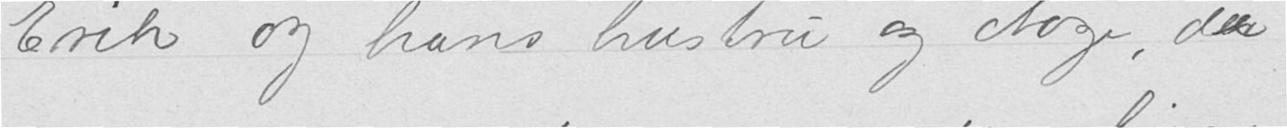Erik og hans hustru og Aage, der
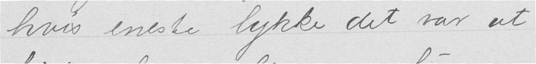hvis eneste lykke det var at
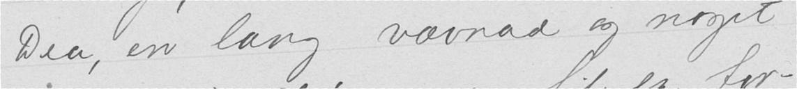Dea, en lang vævnad og noget
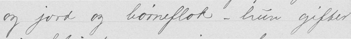og jord og børneflok - hun gifter
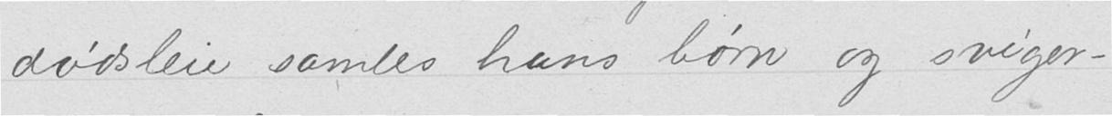dødsleie samles hans børn og sviger-
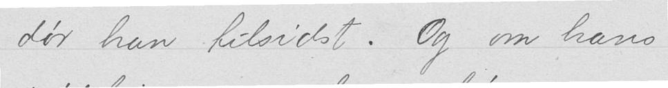dør han tilsidst. Og om hans
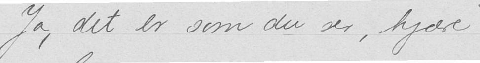Ja, det er som du ser, kjære
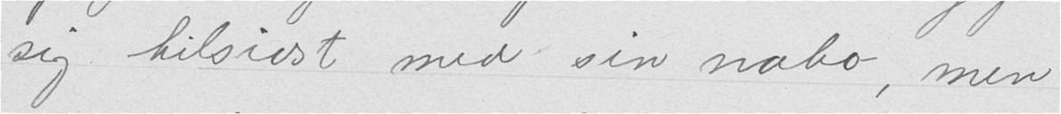sig tilsidst med sin nabo, men
